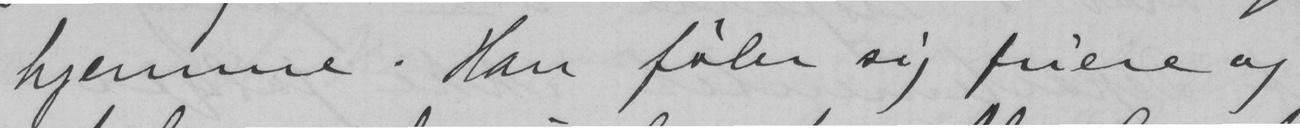hjemme. Han føler sig friere og
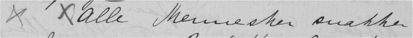x x Alle mennesker snakker
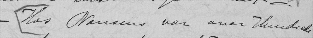- Hos Nansens var over Hundrede
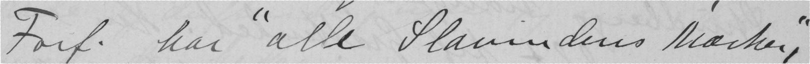Forf. har "alle Slavindens Mærker",
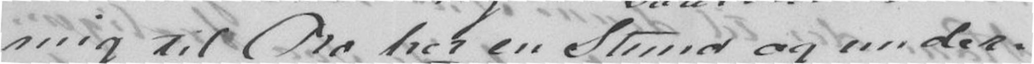mig til Ro her en Stund og under-
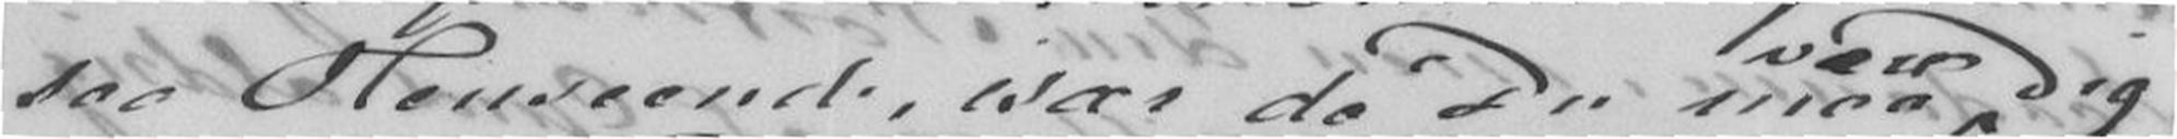saa Henseende især de Du maa Dig
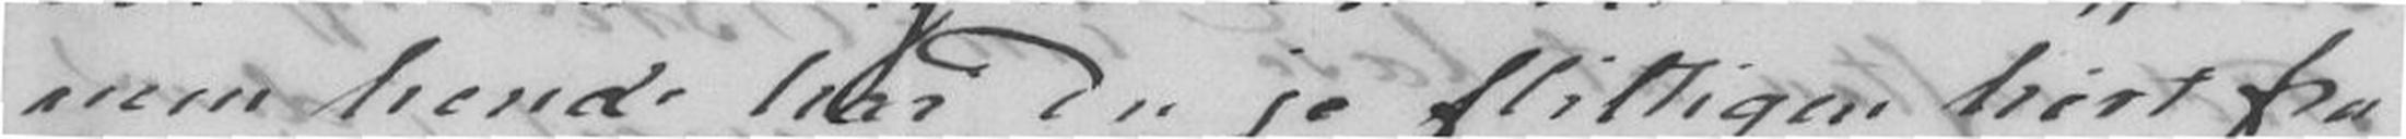men hende har Du jo flittigen hørt fra
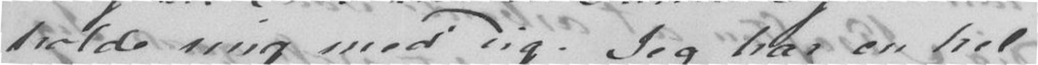holde mig med Dig. Jeg har en hel
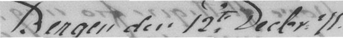Bergen den 12 Decbr. 71
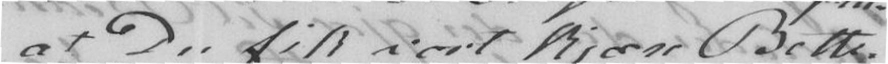at Du fik vort kjære Bette-
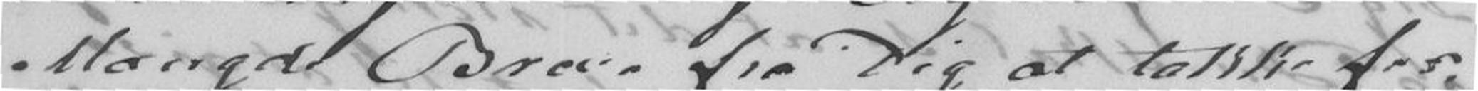Mængde Breve fra Dig at takke for,
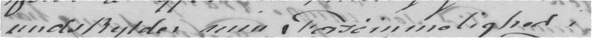undskylder min Forsømmelighed i
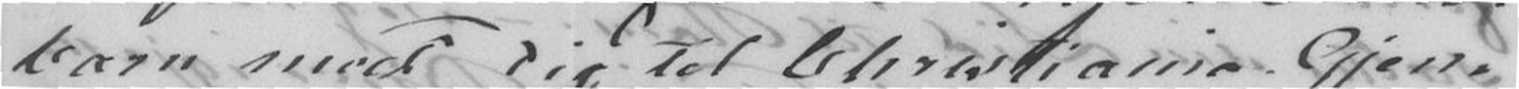barn med Dig til Christiania Gjen-
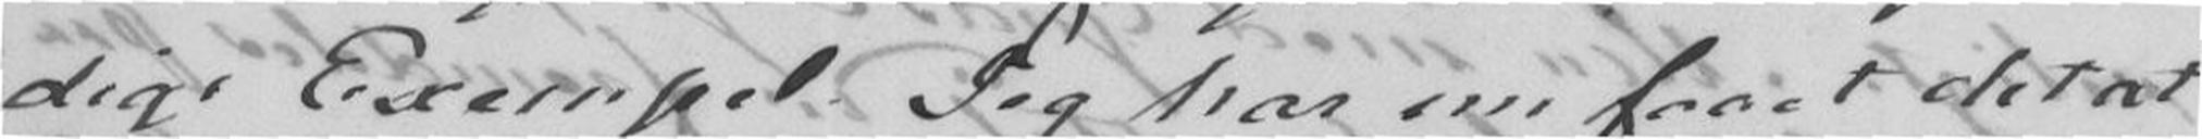dige Exempel. Jeg har nu faaet det at
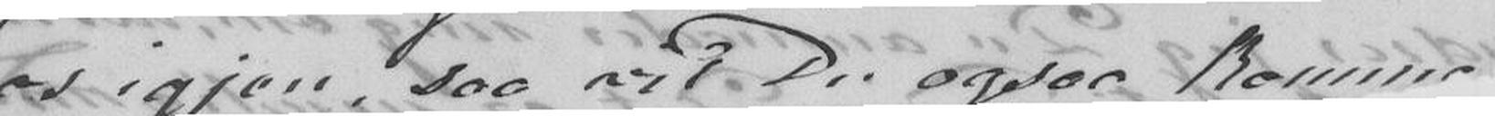os igjen, saa vil Du ogsaa komme
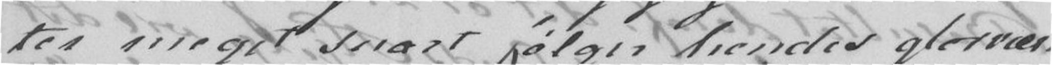ter meget snart følger hendes glorvær-
